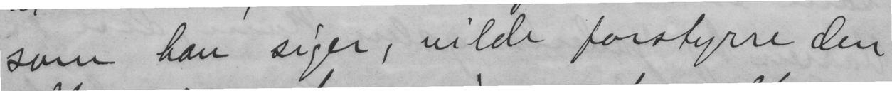som han siger, vilde forstyrre den
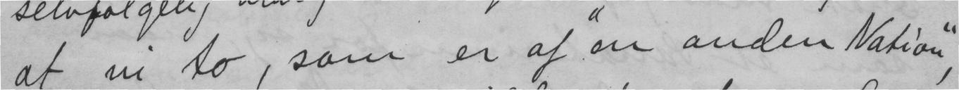at vi to, som er af "en anden Nation",
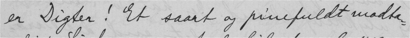er Digter! Et saart og pinefuldt modta-
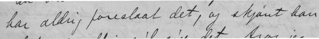har aldrig foreslaat det, og skjønt han
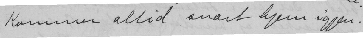Kommer altid snart hjem igjen.
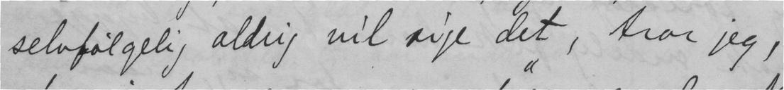selvfølgelig aldrig vil sige det, tror jeg,
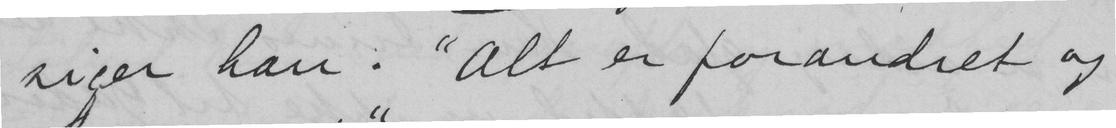siger han. "Alt er forandret og
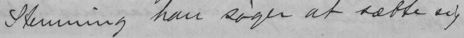Stemning han søger at sætte sig
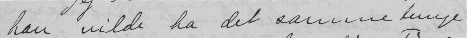han vilde ha det samme tunge
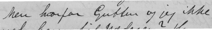Men hvorfor Gutten og jeg ikke
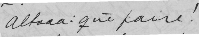Altsaa: que faire!
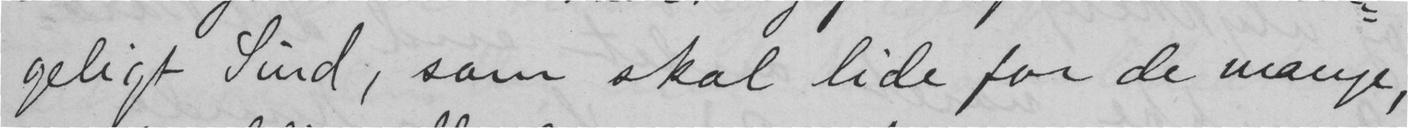geligt Sind, som skal lide for de mange,
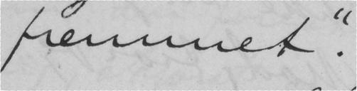fremmet."
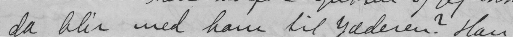da blir med ham til Jæderen? Han
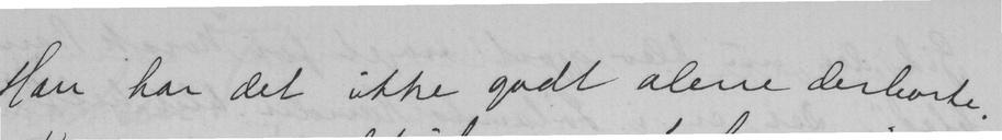Han har det ikke godt alene derborte.
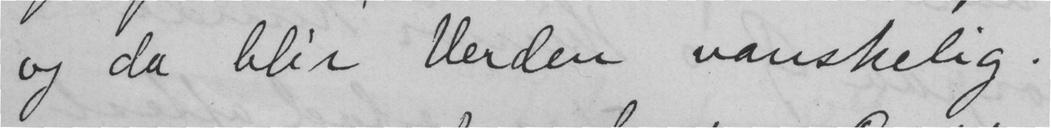og da blir verden vanskelig.
